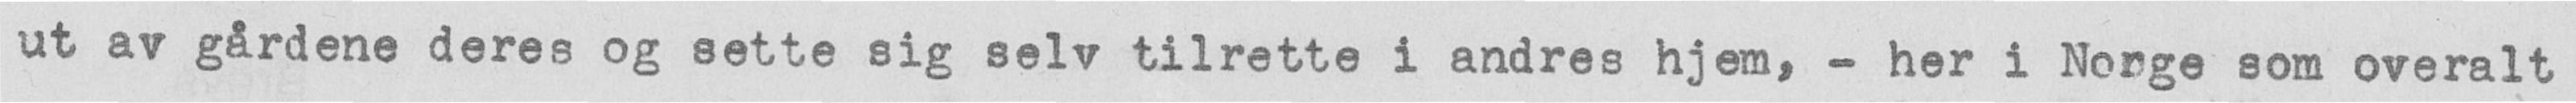ut av gårdene deres og sette sig selv tilrette i andres hjem, - her i Nonge som overalt
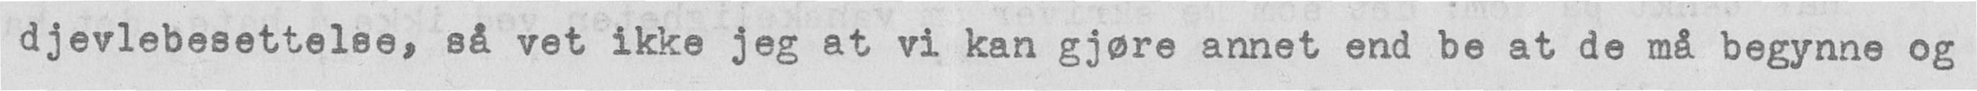djevlebesettelse, så vet ikke jeg at vi kan gjøre annet end be at de må begynne og
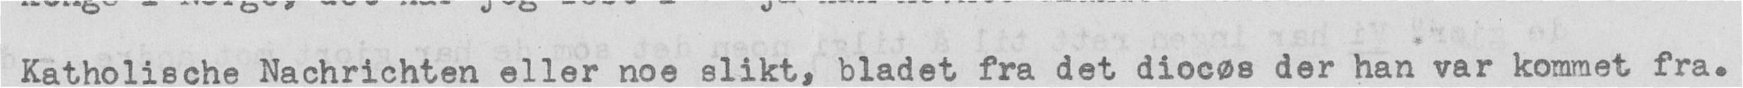Katholische Nachrichten eller noe slikt, bladet fra det diocøs der han var kommet fra.
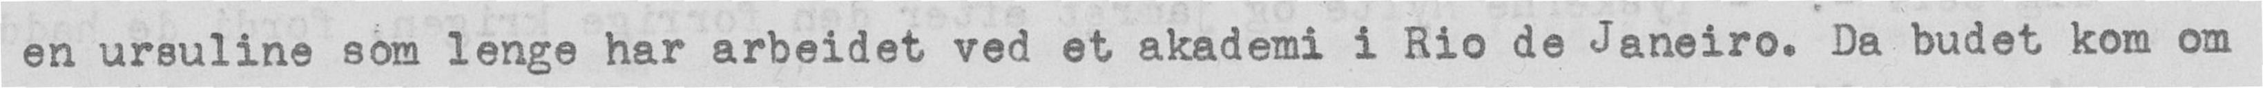en ursuline som lenge har arbeidet ved et akademi i Rio de Janeiro. Da budet kom om
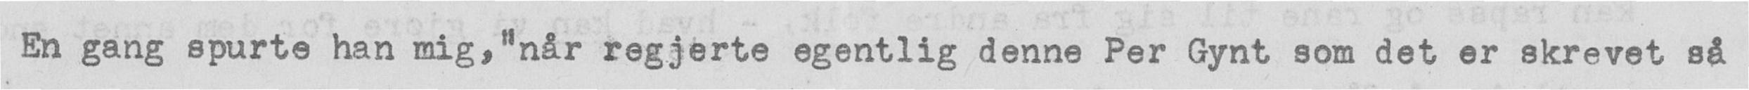En gang spurte han mig,"når regjerte egentlig denne Per Gynt som det er skrevet så
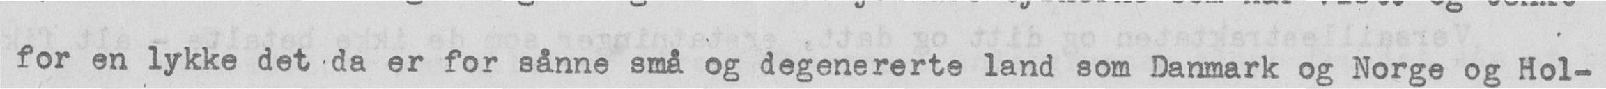for en lykke det da er for sånne små og degenererte land som Danmark og Norge og Hol¬
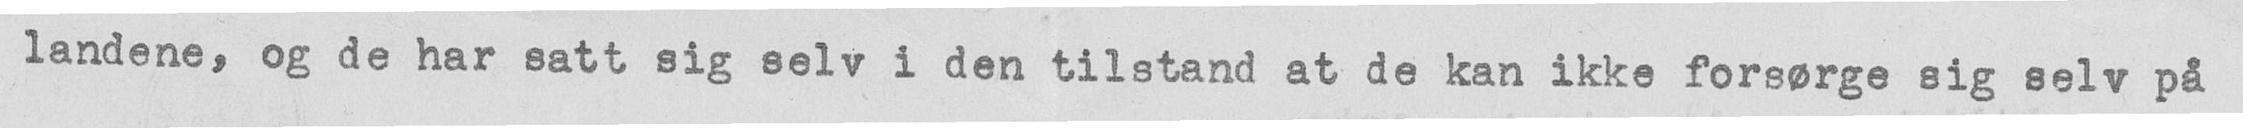landene, og de har satt sig selv i den tilstand at de kan ikke forsørge sig selv på
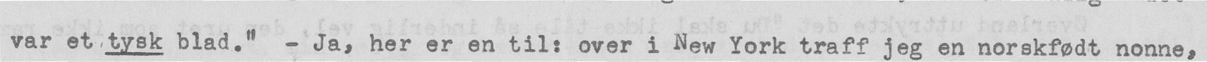var et tysk blad." — Ja, her er en til: over i New York traff jeg en norskfødt nonne,
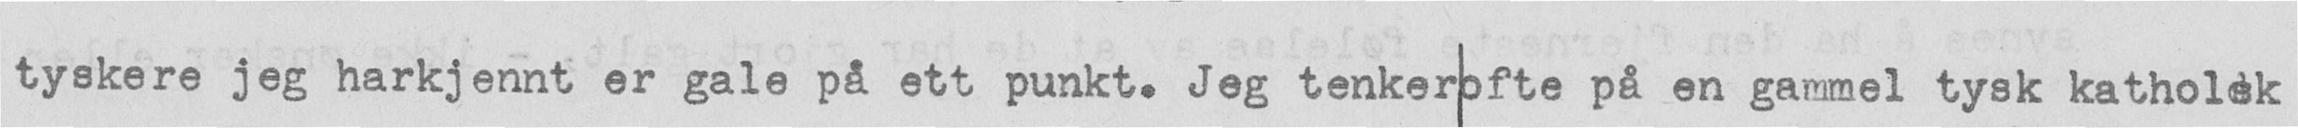tyskere jeg harkjennt er gale på ett punkt. Jeg tenkerofte på en gammel tysk katholek
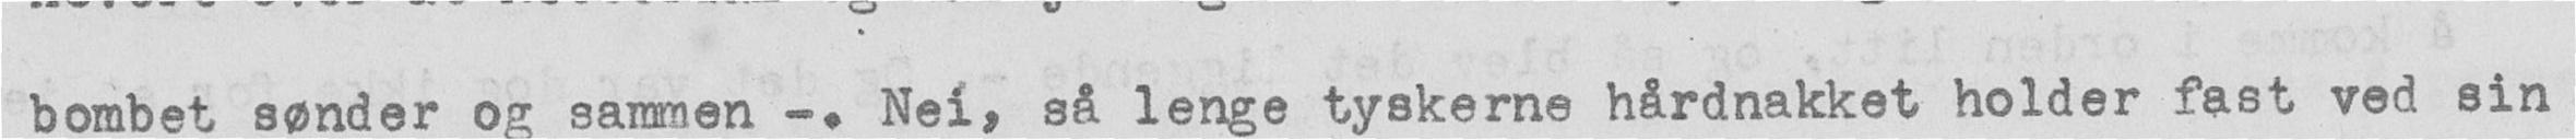bombet sønder og sammen -. Nei, så lenge tyskerne hårdnakket holder fast ved sin
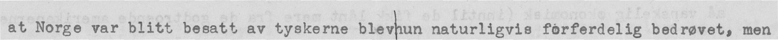at Norge var blitt besatt av tyskerne blevhun naturligvis forferdelig bedrøvet, men
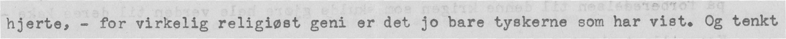hjerte, - for virkelig religiøst geni er det jo bare tyskerne som har vist. Og tenkt
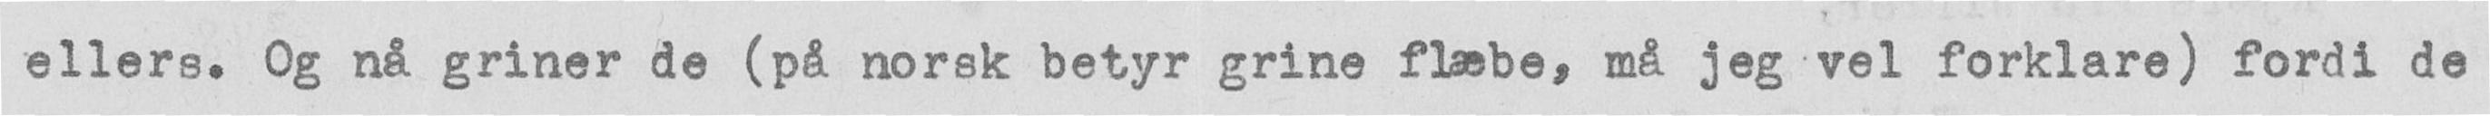ellers. Og nå griner de (på norsk betyr grine flæbe, må jeg vel forklare) fordi de
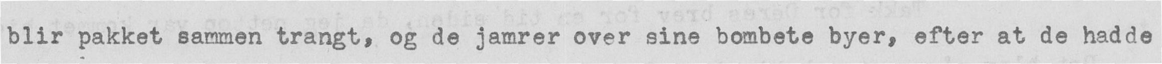blir pakket sammen trangt, og de jamrer over sine bombete byer, efter at de hadde
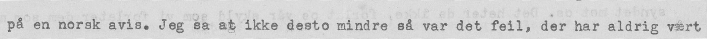på en norsk avis. Jeg sa at ikke desto mindre så var det feil, der har aldrig vært
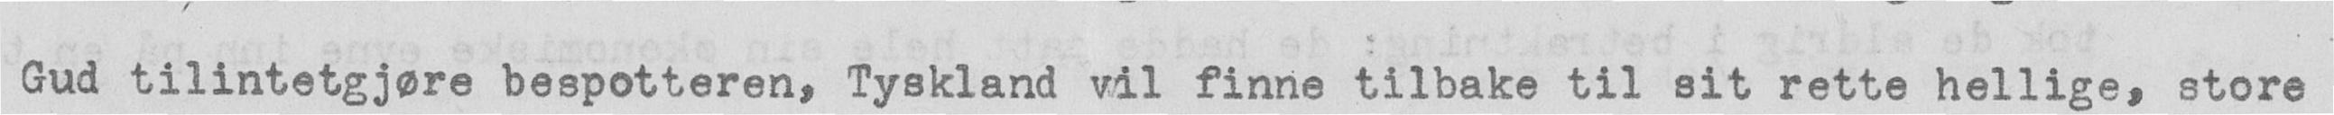Gud tilintetgjøre bespotteren, Tyskland vil finne tilbake til sit rette hellige, store
